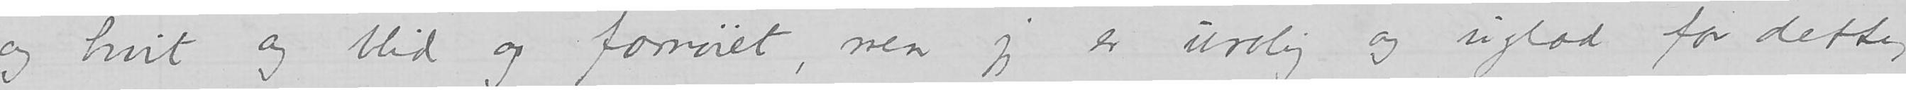og blid og fornøiet, men jeg er urolig og uglad for dette,
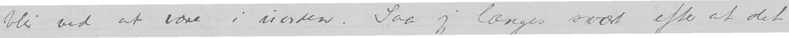blir ved at være i uorden. Saa jeg længes svært efter at det
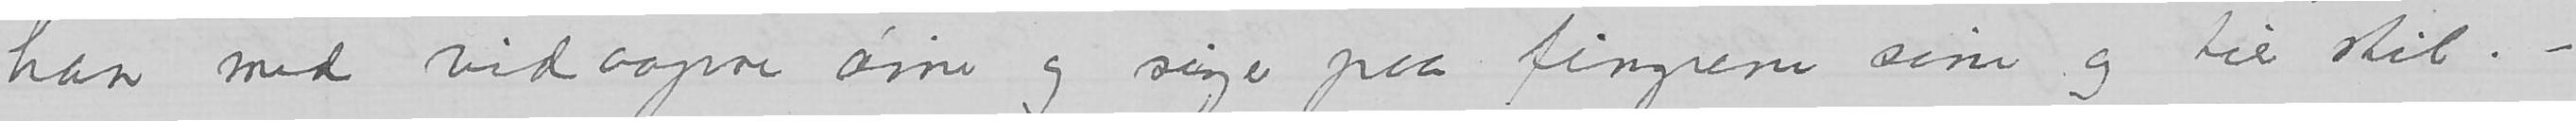han med vidaapne øine og suger paa fingrene sine og tier stil. –
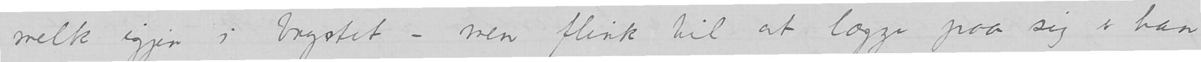melk igjen i brystet – men flink til at lægge paa sig er han
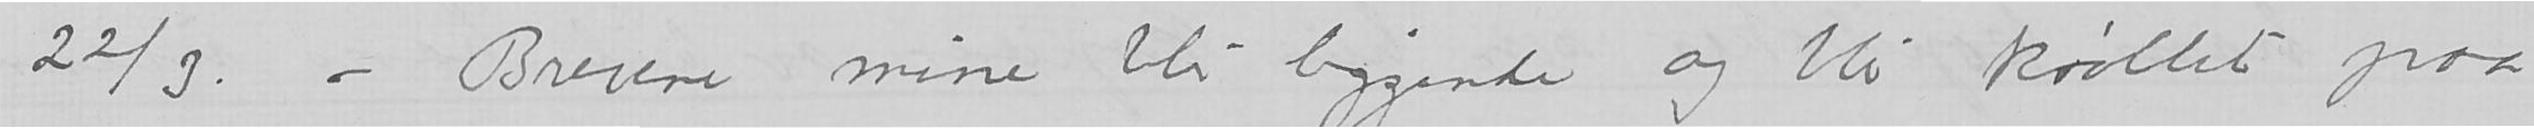22/3. – Brevene mine blir liggende og blir krøllet paa
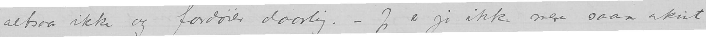altsaa ikke og fordøier daarlig. – Jeg er ikke mere saan akut
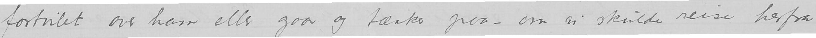fortvilet over ham eller gaar og tænker paa – om vi skulde reise herfra
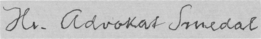Hr. Advokat Smedal
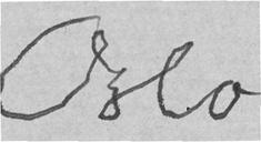Oslo
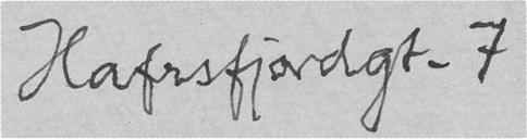Hafrsfjordgt. 7
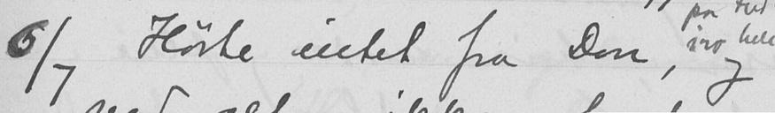6/7 Hørte intet fra Don, og
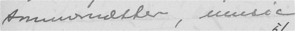sommernætter, music
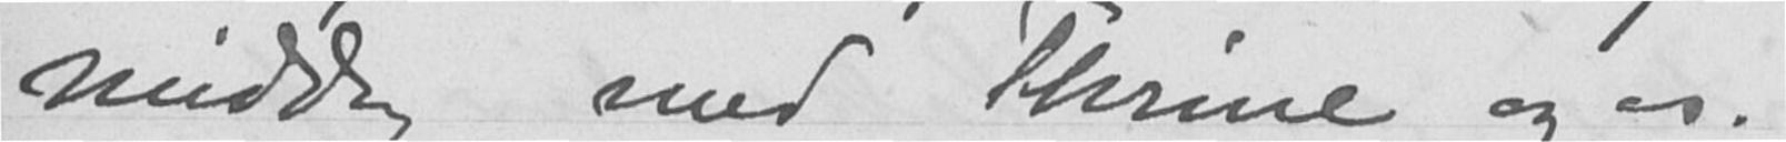middag med Thrine og os.
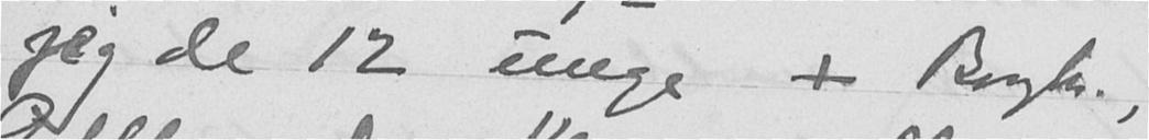jeg de 12 unge + Borgh.,
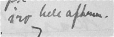i ro hele aftenen.
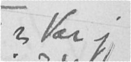? Var jeg
